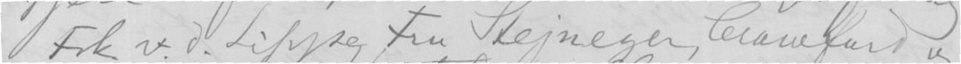Frk v. d. Lippe, Fru Stejmeger, Crawford og
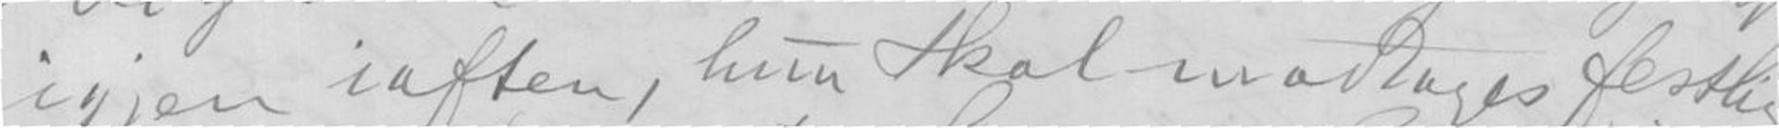igjen iaften, hun skal modtages festlig.
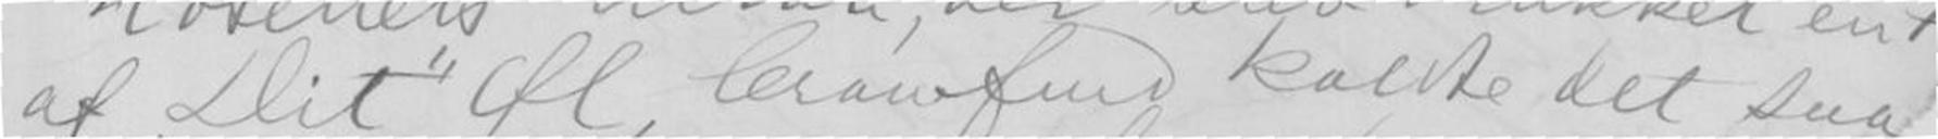af Dit Øl, Crawford kaldte det saa
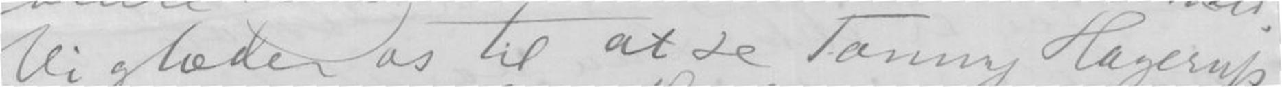Vi glæder os til at se Tonny Hagerup
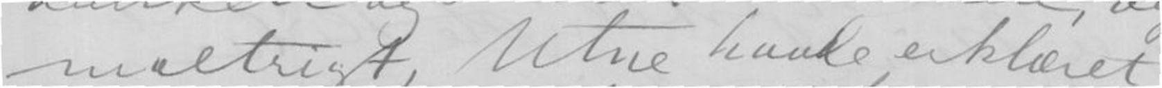maltrigt, Utne havde erklæret
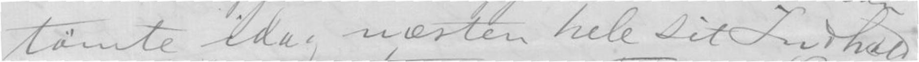tømte idag næsten hele sit Indhold.
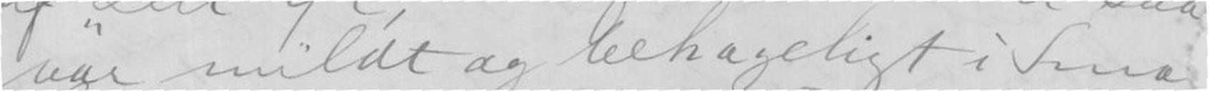var mildt og behageligt i Sma
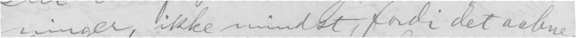ninger, ikke mindst, fordi det aabne-
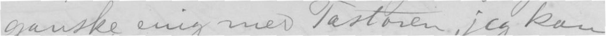ganske enig med Pastoren, jeg kan
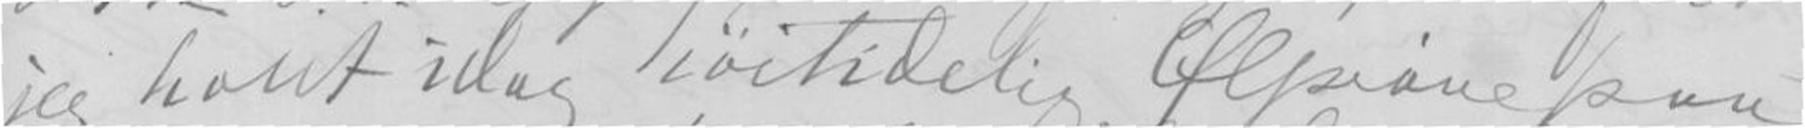jeg havt idag høitidelig Ølprøve paa
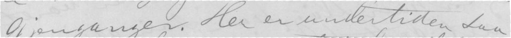Gjenganger. Her er undertiden saa
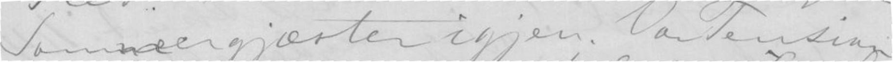Sommergjæster igjen. Vor Pension
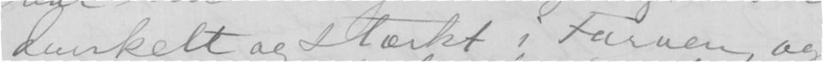dunkelt og stærkt i Farven, og
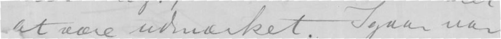at være udmærket. Igaar va
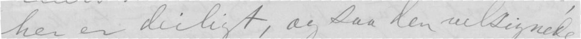her er deiligt, og saa den velsignede
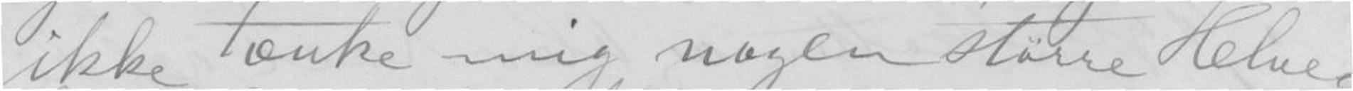ikke tænke mig nogen større Helve-
